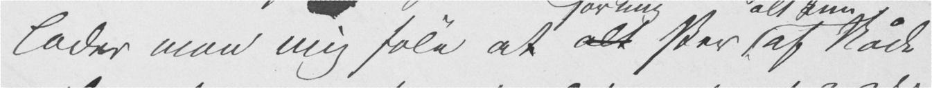lader man mig føle at alt sker af Nåde
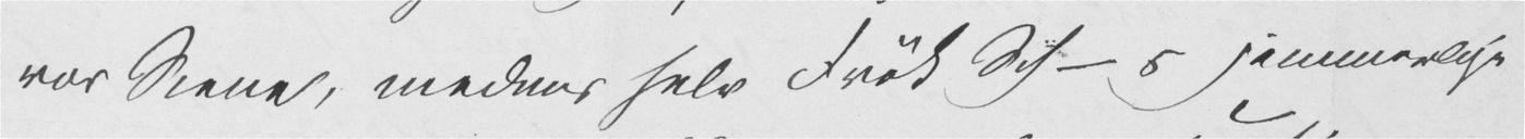vor Scene, medens helv Frøk Sch–s jammerlige
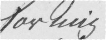for mig
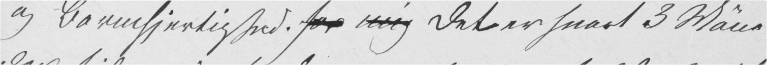og Barmhjertighed. for mig det er snart 3 Måne
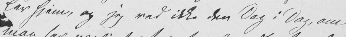ler hjem, og jeg ved ikke den Dag i Dag, om
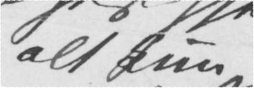alt kun
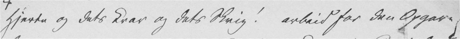Hjerte og dets Krav og dets Skrig! arbeid for den Opgave
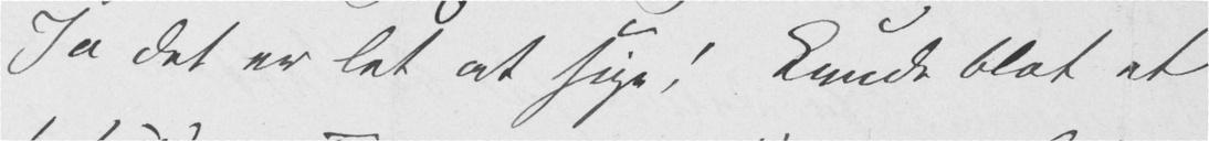Ja det er let at sige! Kunde blot et
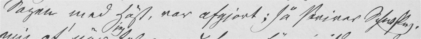Sagen med Høst, var afgjort; så skriver Schwflug,
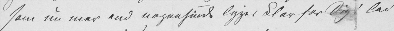som nu mer end nogensinde ligger klar for Dig! lad
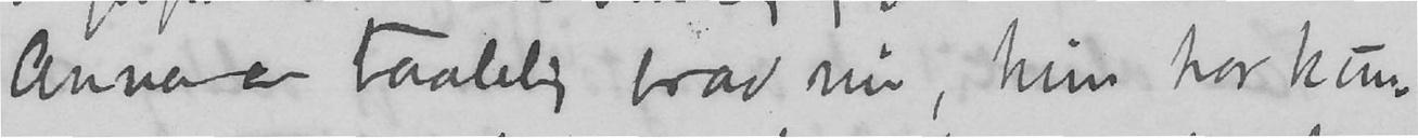Anna er taalelig brav nu, hun har kun-
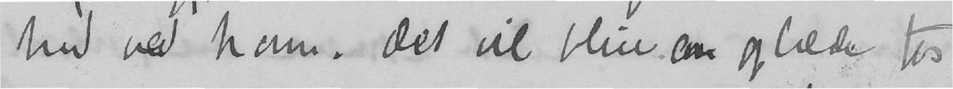hed ved ham. Det vil blive en glæde for
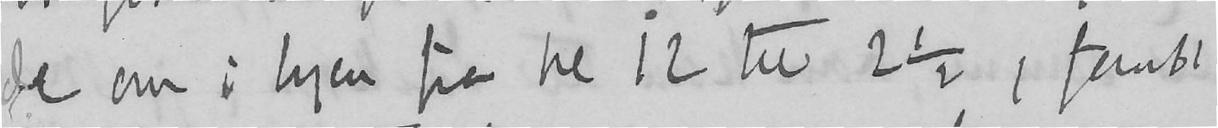de om i byen fra kl 12 til 2½, fandt
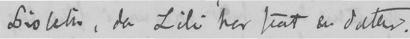Lisbeth, da Lili har faat en datter.
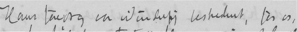Hans foredrag var vidunderlig beskedent, for os,
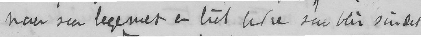naar saa legemet er lidt bedre saa blir sindet
